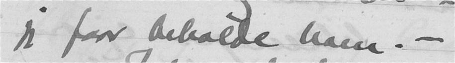jeg faar beholde ham. -
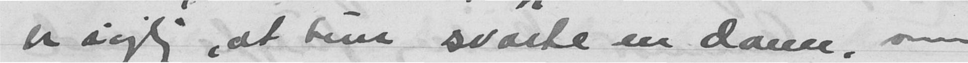er rigtig, at hun svarte en dame, som
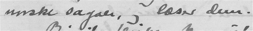norske sagaer, og læser dem.
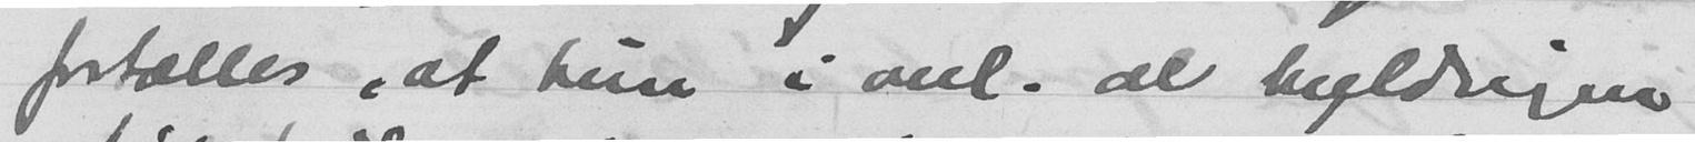fortælles, at hun i anl. al hyldingen
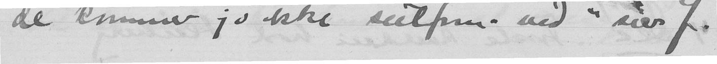de kommer jo ikke seilforn. ved" sier J.
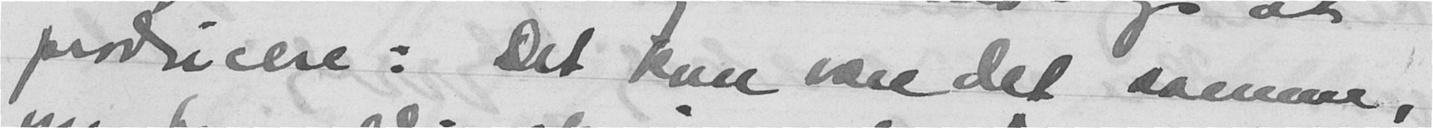producere: Det kan være det samme,
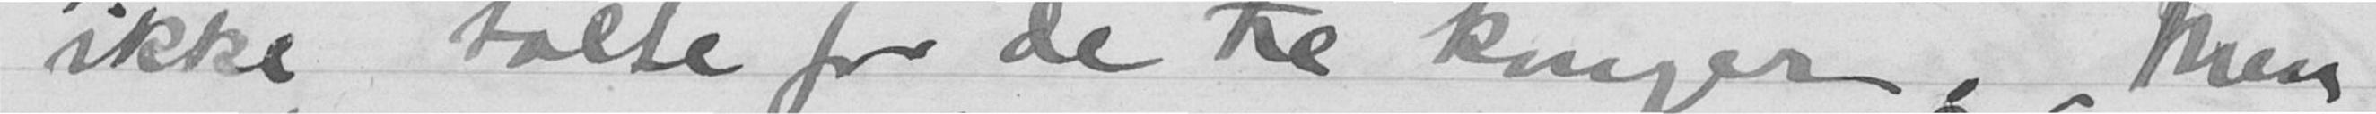ikke talte for de tre konger. "Men
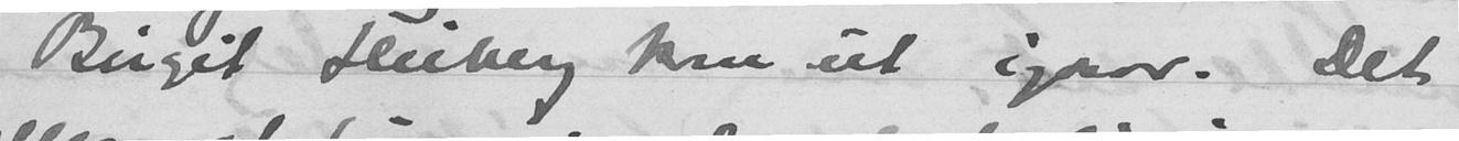Birgit Heiberg kom ut igaar. Det
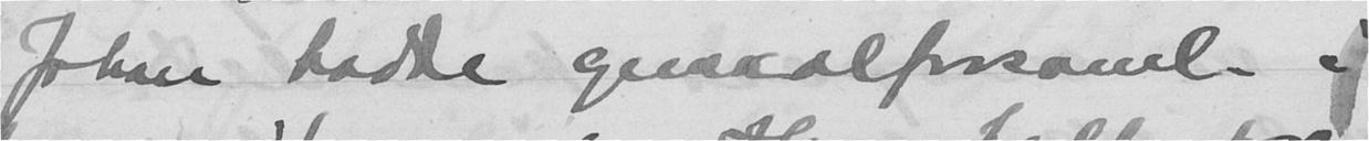Johan hadde generalforsaml. i
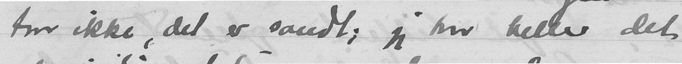tror ikke, det er sandt; jeg tror heller det
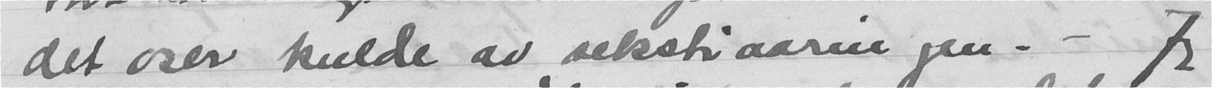det oser kulde av sekstiaaringen. - Jeg
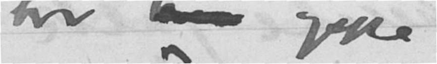har ha oppe
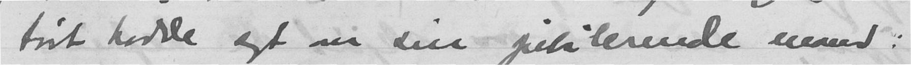først hadde sagt om sin jubilerende mand:
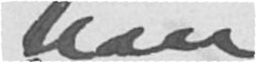han
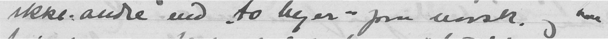ikke andre end "to byer" paa norsk, og han
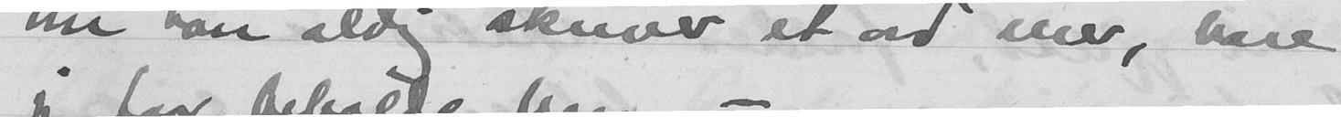om han aldrig skriver et ord mer, bare
